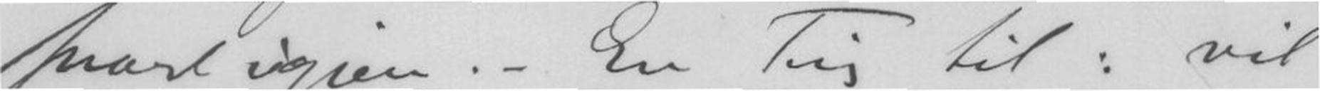snart igjen. - En Ting til: vil
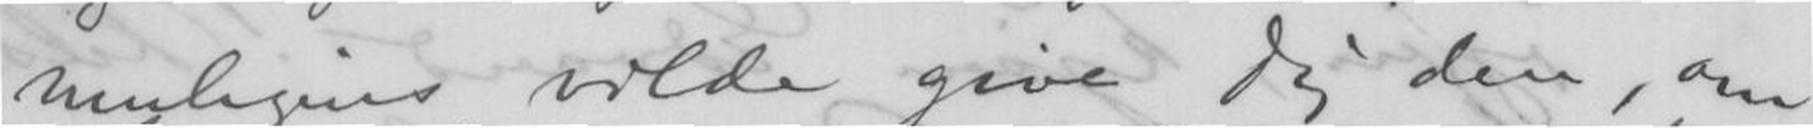muligens vilde give dig den, om
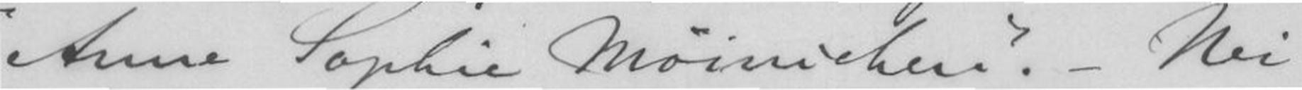"Anne Sophie Møinichen." - Nei
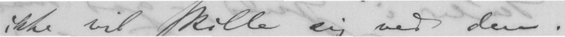ikke vil skille sig ved den.
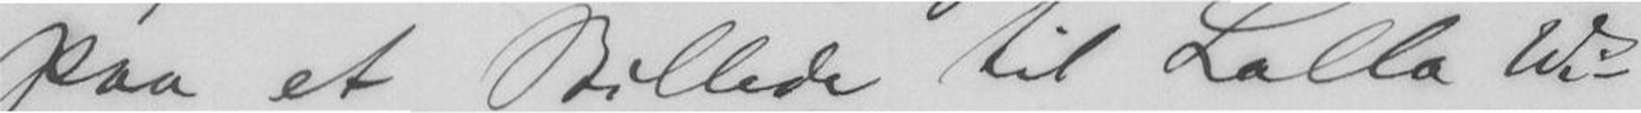paa et Billede til Lalla Wi-
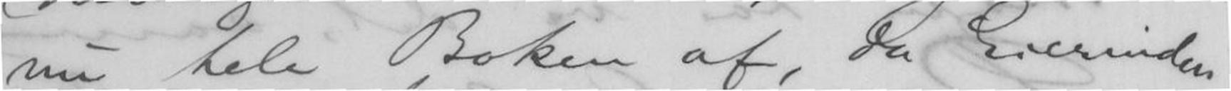nu hele Boken af, da Eierinden
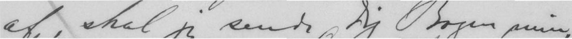af, skal jeg sende dig Bogen min,
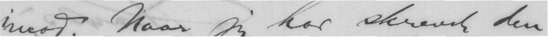imod. Naar jeg har skrevet den
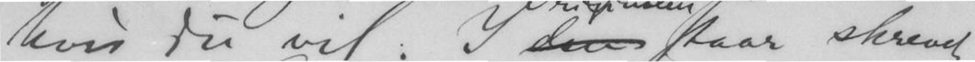hvis du vil. I den staar skrevet
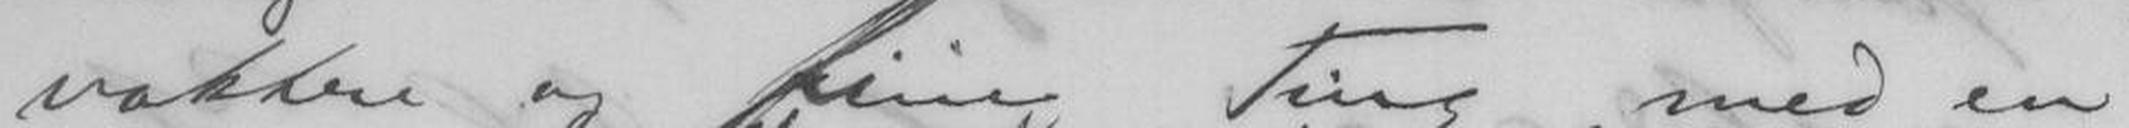vakkre og fine Ting, med en
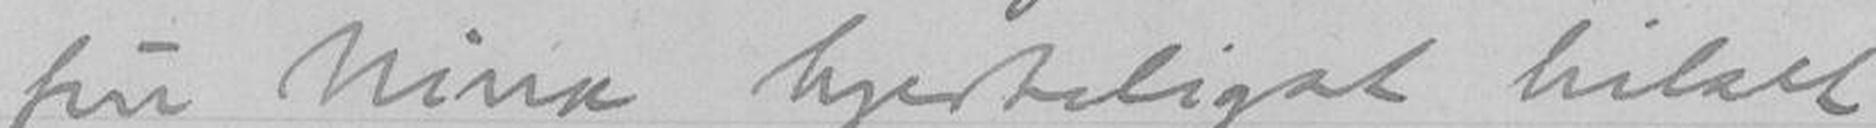Fru Nina hjerteligst hilset
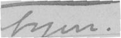byen.
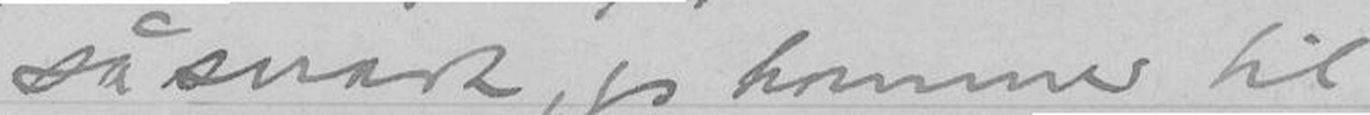så snart, jeg kommer til
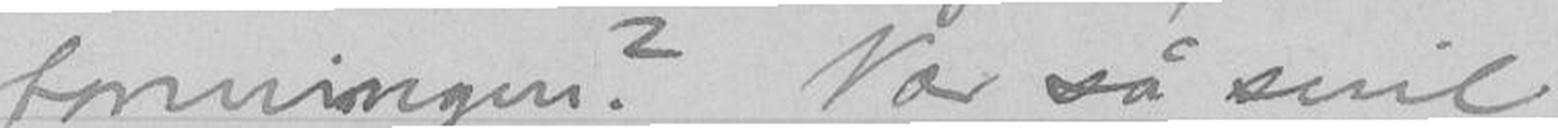foreningen? Vær så snil
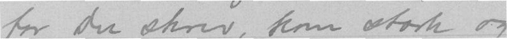for du skrev, kom stærk og
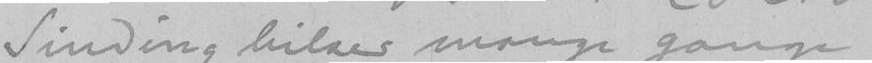Sinding hilser mange ganger.
